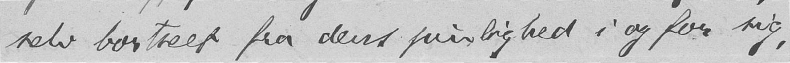selv bortseet fra dens pinlighed i og for sig,
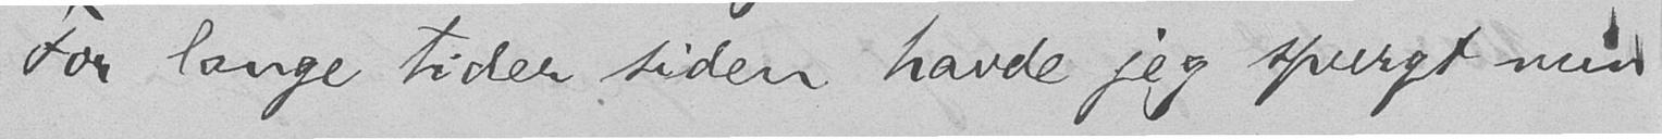For lange tider siden havde jeg spurgt min
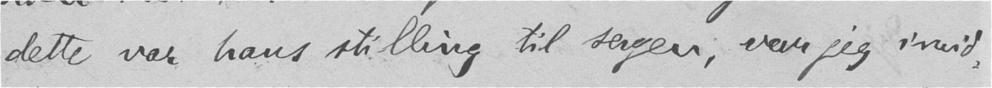dette var hans stilling til sagen, var jeg imid-
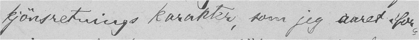kjønsretnings karakter, som jeg aaret ifor-
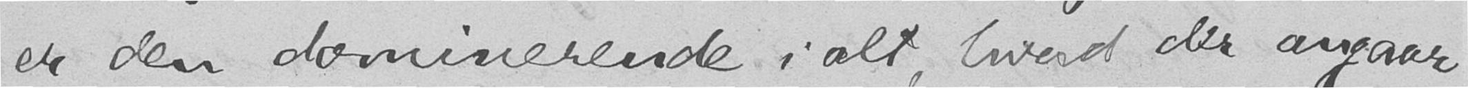er den dominerende i alt, hvad der angaar
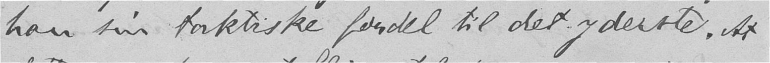han sin taktiske fordel til det yderste. At
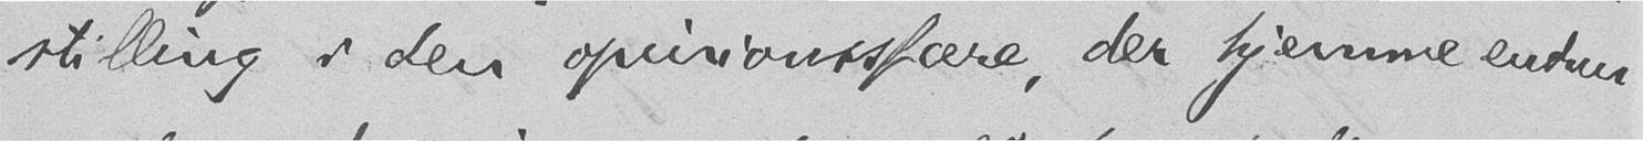stilling i den opinionssfære, der hjemme endnu
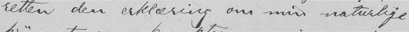retten den erklæring om min naturlige
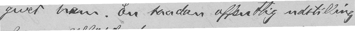givet ham. En saadan offentlig udstilling
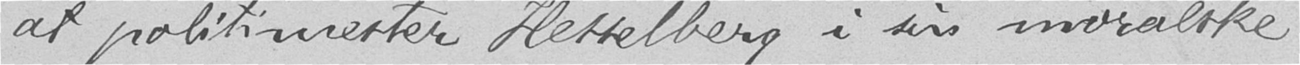at politimester Hesselberg i sin moralske
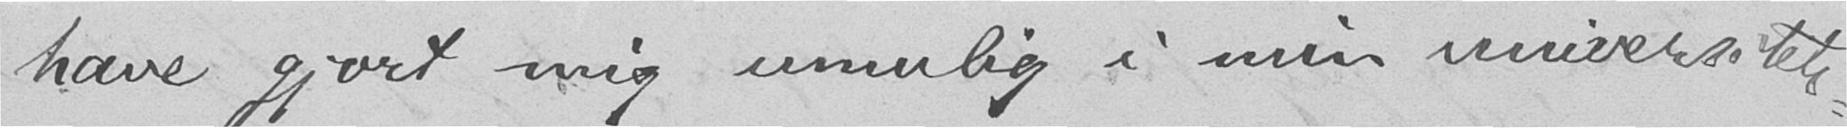have gjort mig umulig i min universitets-
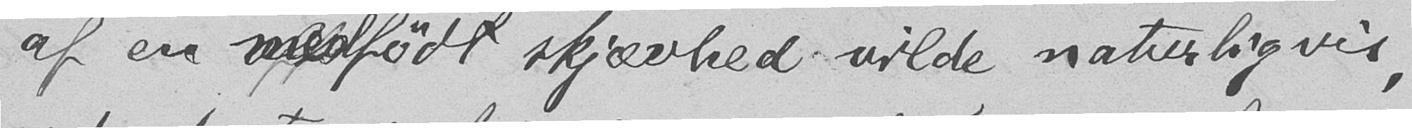af en medfødt skjævhed vilde naturligvis,
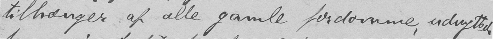tilhænger af alle gamle fordomme, udnyttede
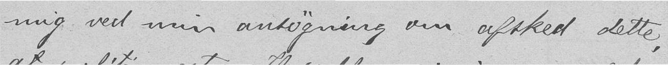mig ved min ansøgning om afsked dette,
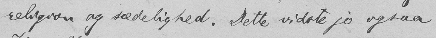religion og sædelighed. Dette vidste jo ogsaa
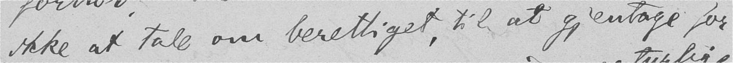ikke at tale om berettiget, til at gjentage for
